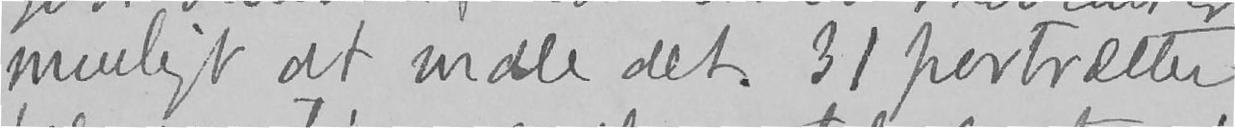muligt at male det. 31 portrætter,
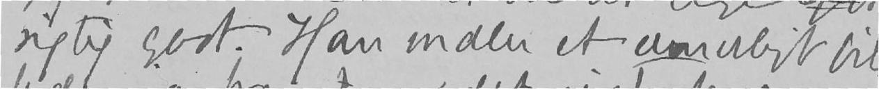rigtig godt. Han maler et umuligt bil-
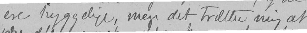ere hyggelige, men det trætter mig at
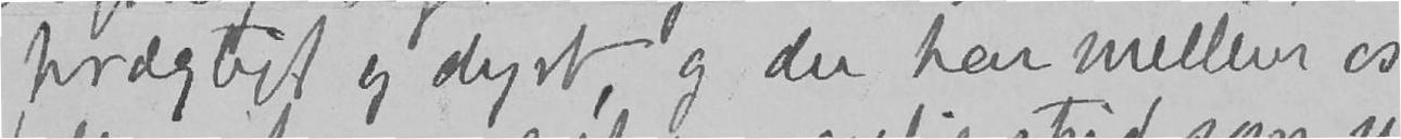kvægbif og dyrt, og der har mellem os
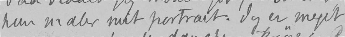hun maler mit portrait. Jeg er meget
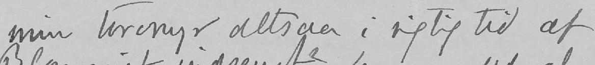min torvmyr altsaa i rigtig tid af
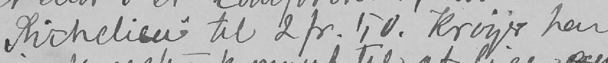Richelieu til 2 fr. 50. Krøyer har
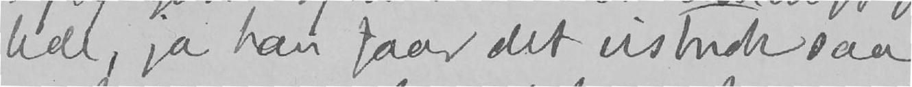lede, ja han faar det vistnok saa
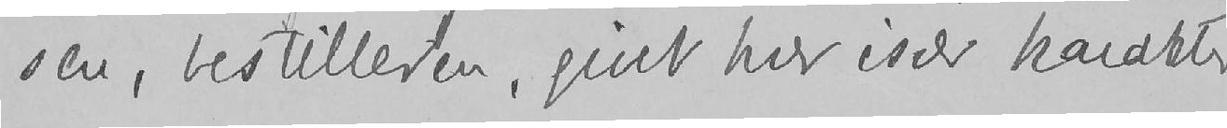sen, bestilleren, givet hver især karakter
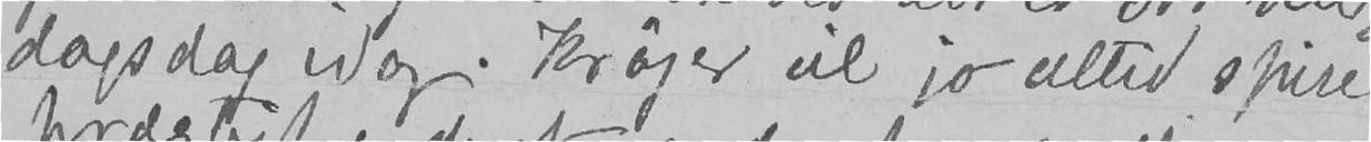ddagsdag idag. Krøyer vil jo altid spise
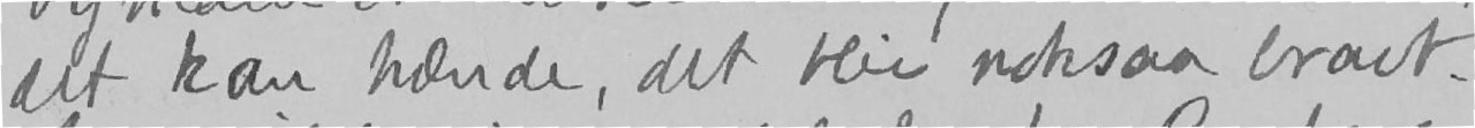det kan hænde, det blir noksaa bravt.
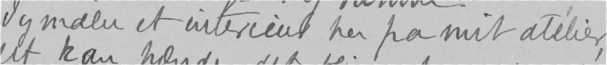Jeg maler et interieur her fra mit atélier,
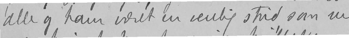alle og ham været en venlig strid som nu
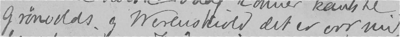Grønvolds og Werenskiold det er vor mi-
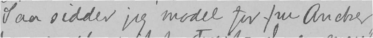Saa sidder jeg model for fru Ancker,
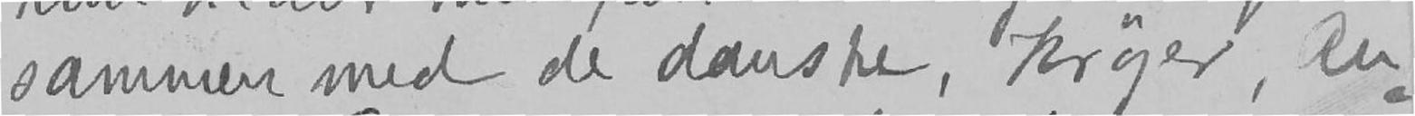sammen med de danske, Krøyer, An-
